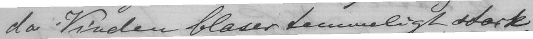da Vinden blæser temmeligt stærkt.
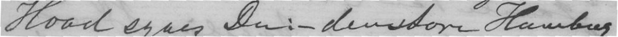Hvad synes Du - denstore Hambug
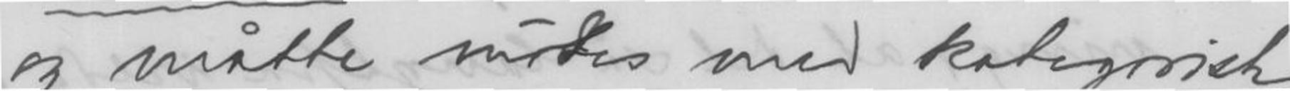og måtte mødes med kategorisk
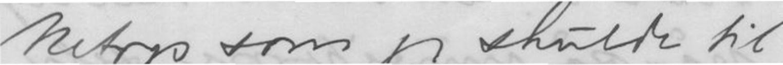Netop som jeg skulde til
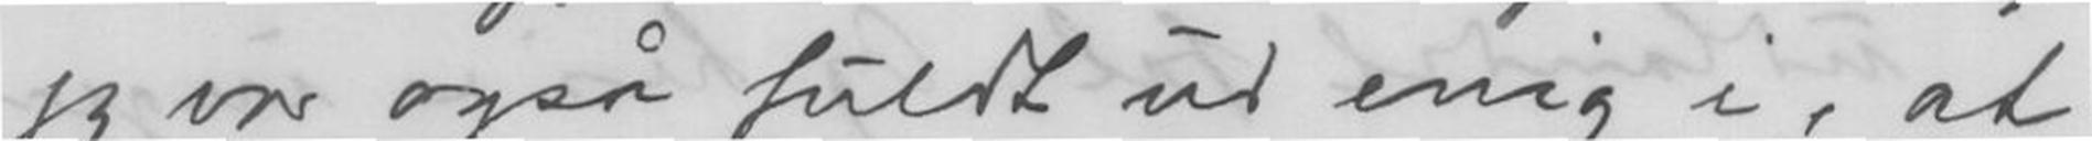jeg var også fuldt ud enig i, at
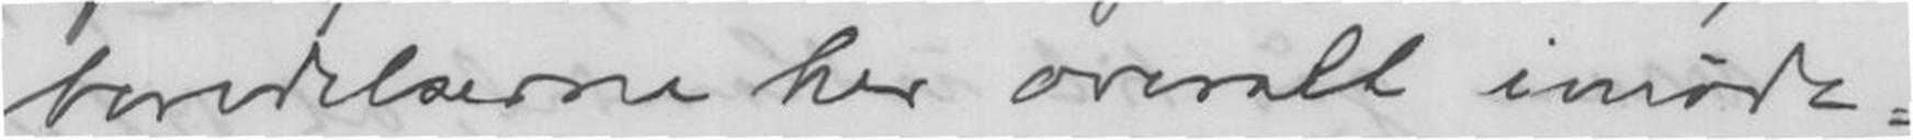beredelserne her overalt imøde-
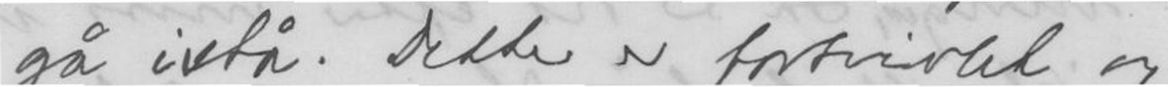gå istå. Dette er fortvilet og
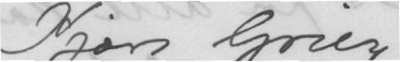Kjære Grieg!
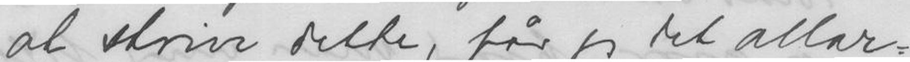at skrive dette, får jeg det allar-
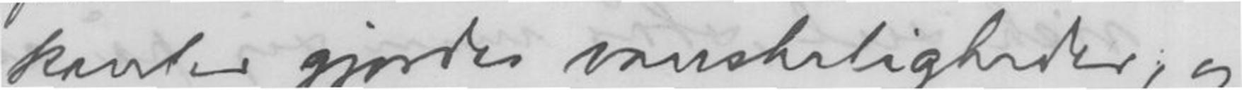kanter gjordes vanskeligheder, og
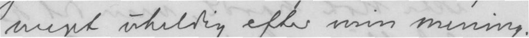meget uheldig efter min mening.
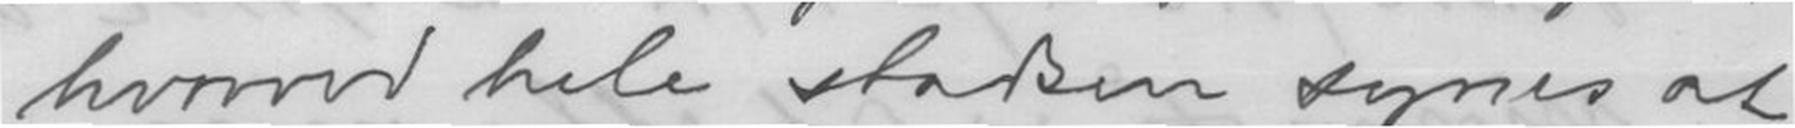hvorved hele stadsen synes at
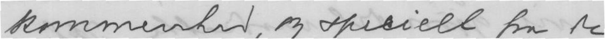kommenhed, og specielt fra de
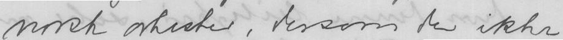norsk orkester, dersom der ikke
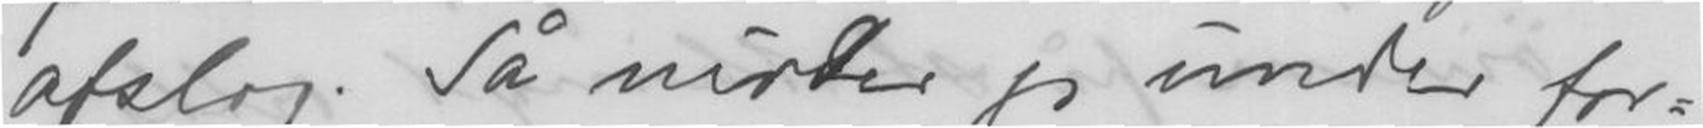afslag. Så møder jeg under for-
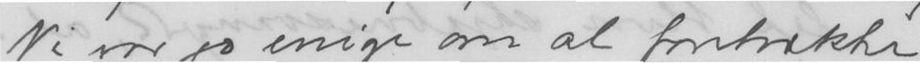Vi var jo enige om at foretrække
